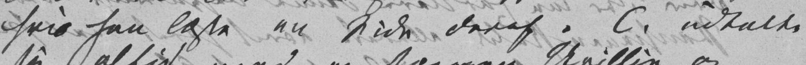hvis han læste en Side deraf. C. udtalte
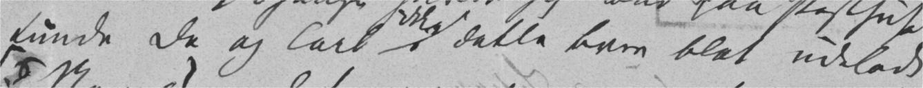Kunde De og Carl ikke i dette Brev blot udeladt
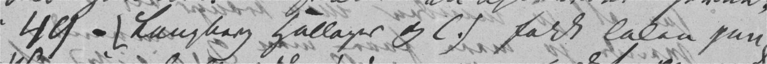i 49 – (Langberg Hallager og C.) faldt Talen paa
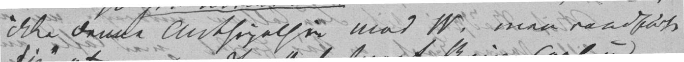ikke denne Anthipathie mod W, men raadførte
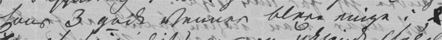hans 3 gode Venner bleve enige i 2
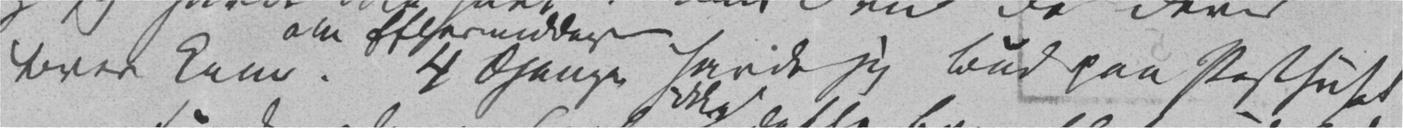kom om Eftermiddagen. 4 Gange havde jeg Bud paa Posthuset
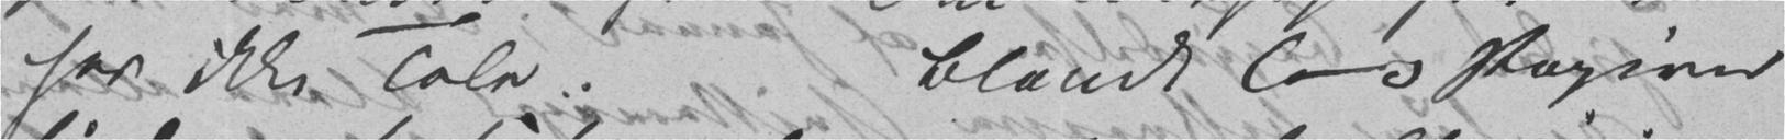her ikke Tale. Blandt C-s Papirer
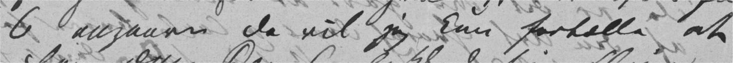C angaaer da vil jeg kun fortælle at
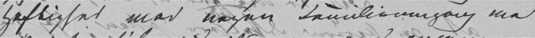Heftighed mod nogen Familieomgang med
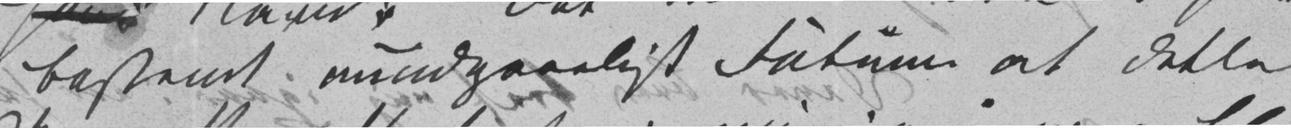bestemt uundgaaeligt Fatum at dette
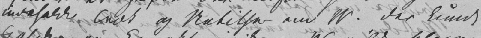indeholder Træk og Notitser om W. der kunde
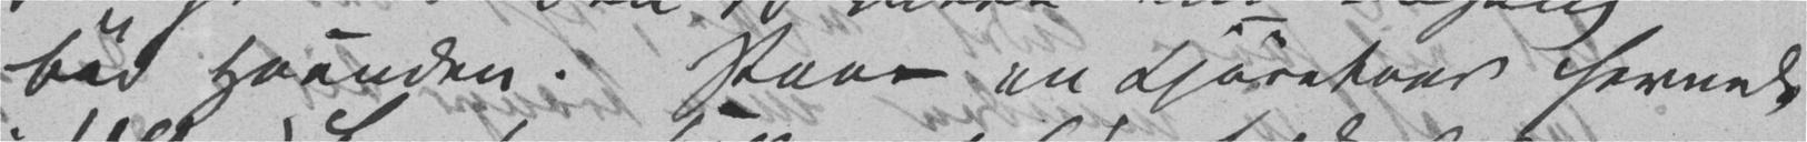bød Haanden. Paa en Kjøretour hernede
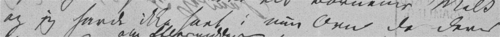og jeg havde ikke havt i min Ovn da Deres Brev
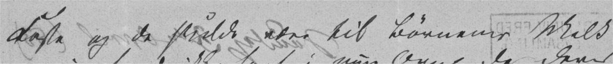Kasse og de skulde være til Børnenes Melk
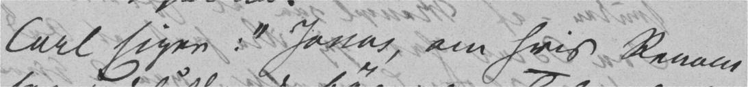Carl siger: «Jonas, om hvis Renom
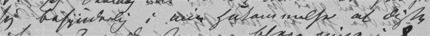sig besynderlig i min Hukommelse at disse
